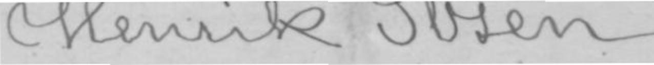Henrik Ibsen.
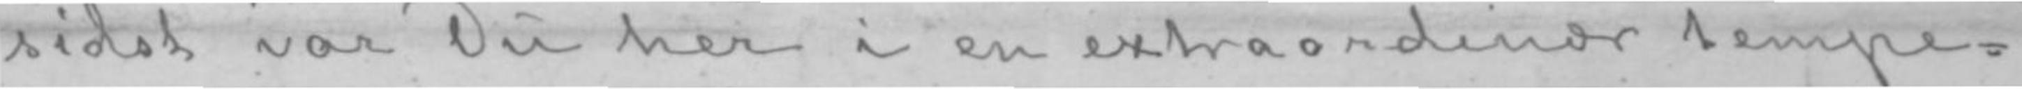sidst var Du her i en extraordinær tempe-
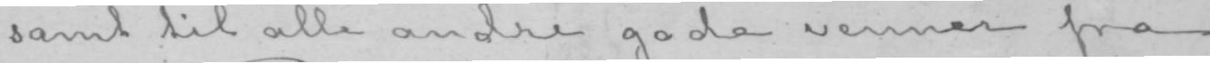samt til alle andre gode venner fra
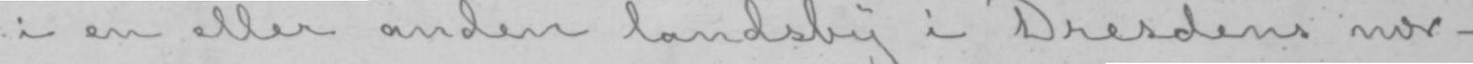i en eller anden landsby i Dresdens nær-
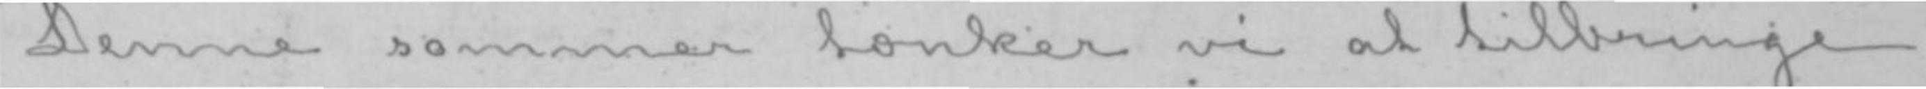Denne sommer tænker vi at tilbringe
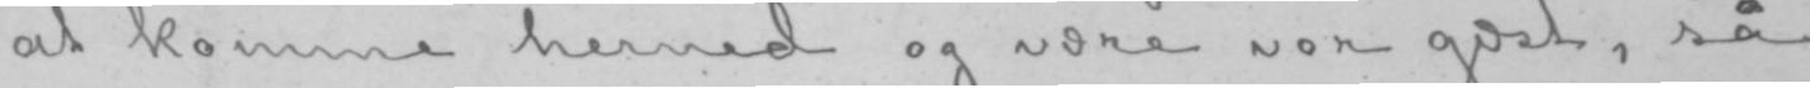at komme herned og være vor gæst, så
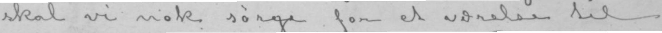skal vi nok sørge for et værelse til
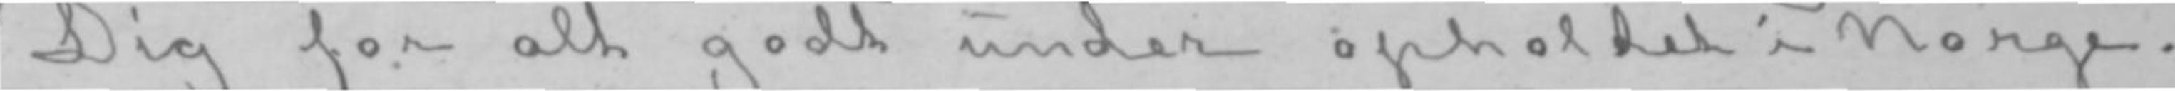Dig for alt godt under opholdet i Norge.
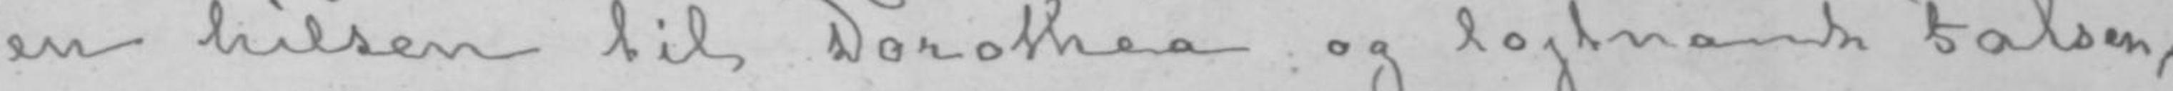en hilsen til Dorothea og løjtnant Falsen,
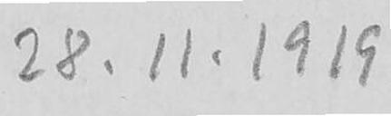28.11.1919
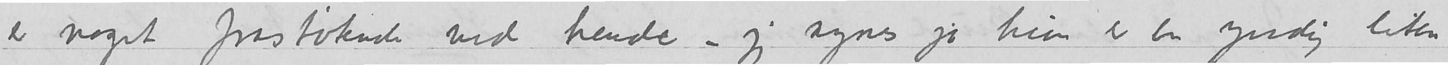er noget frastøtende ved hende – jeg synes jo hun er en yndig liten
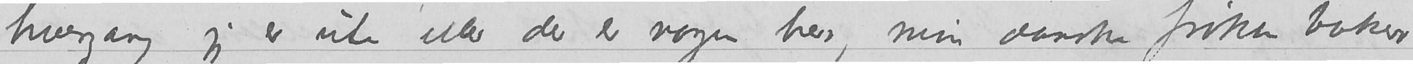hvergang jeg er ute eller der er nogen her, min danske frøken baker
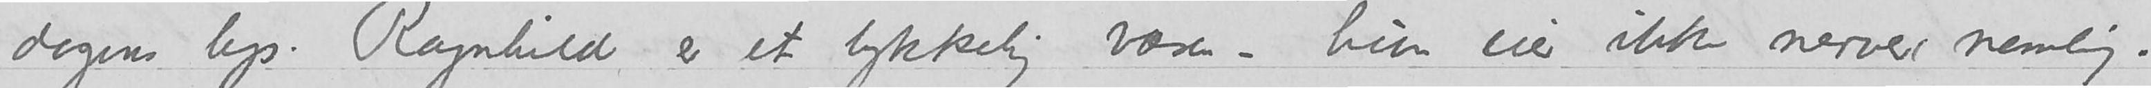dagens lys. Ragnhild er et lykkelig væsen – hun eier ikke nerver nemlig.
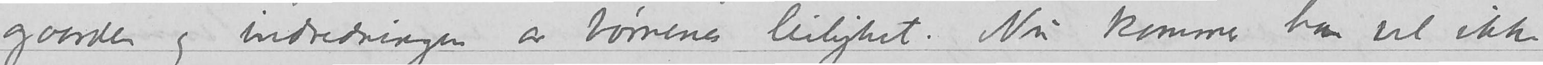gaarden og indredningen av børnenes leilighet. Nu kommer han vel ikke
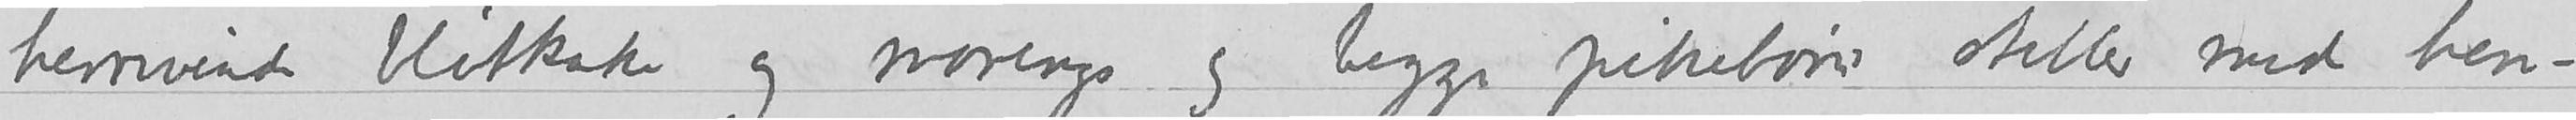henrivende bløtkake og marengs og begge pikebørn steller med hen-
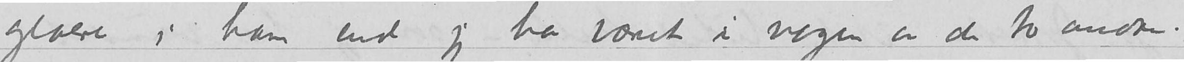glaere i ham end jeg har været i nogen av de to andre.
